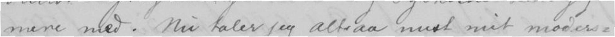mere med. Nu taler jeg altsaa mest mit moders-
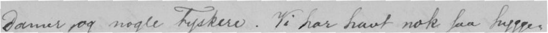Damer og nogle Tyskere. Vi har havt noksaa hygge-
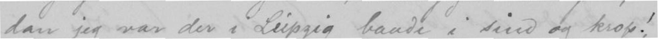dan jeg var der i Leipzig baade i sind og krop!
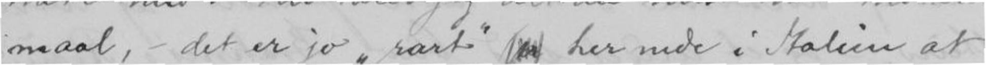maal, - det er jo "rart" her nede i Italien at
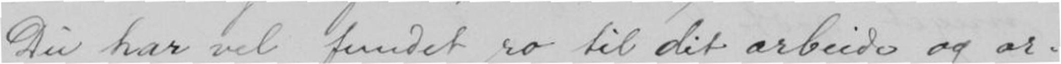Du har vel fundet ro til arbeide og ar-
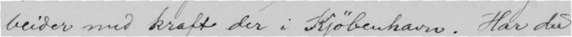beider med kraft der i Kjøbenhavn. Har du
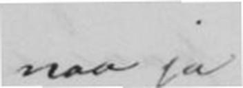naa ja
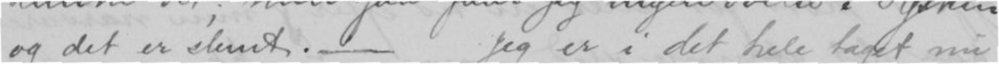og det er slemt. - Jeg er i det hele taget nu
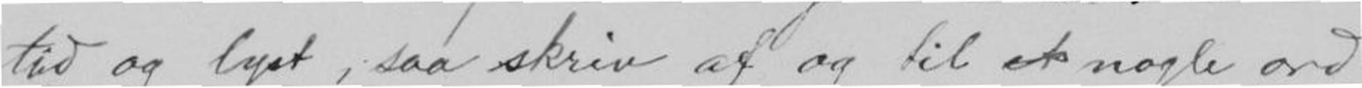tid og lyst, saa skriv af og til nogle ord
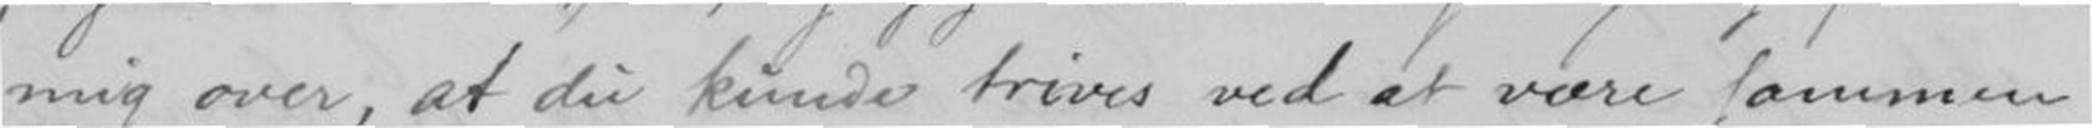mig over, at du kunde trives ved at være sammen
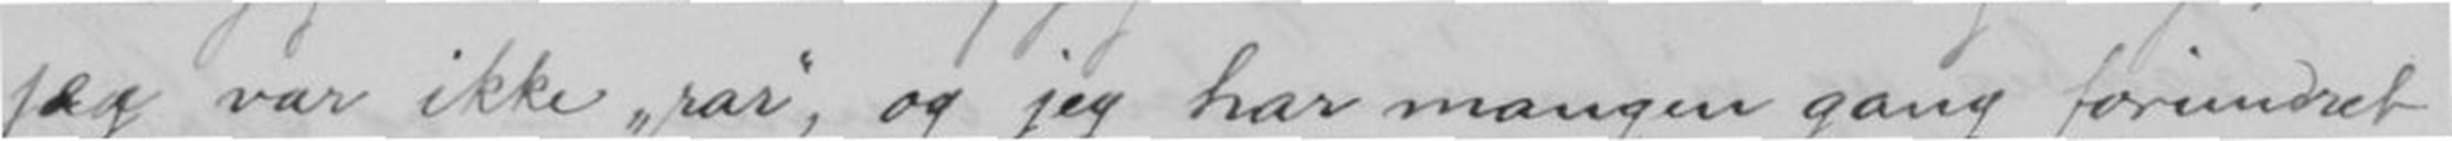Jeg var ikke "rar", og jeg har mangen gang forundret
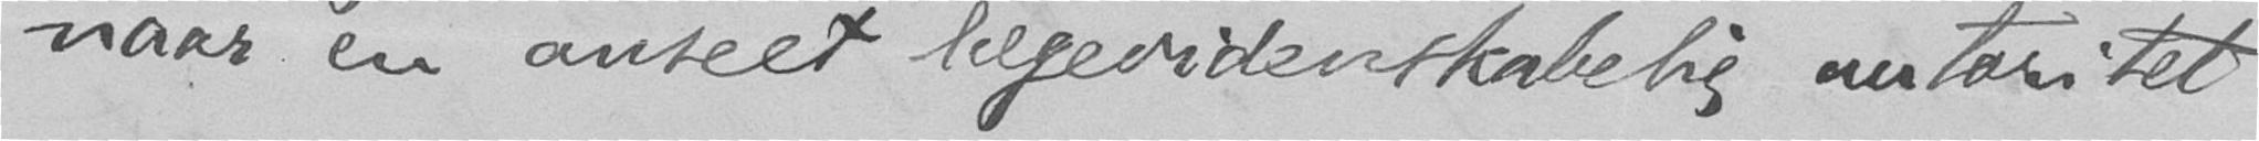naar en anseet lægevidenskabelig autoritet
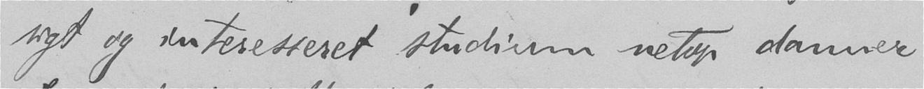sigt og interesseret studium netop danner
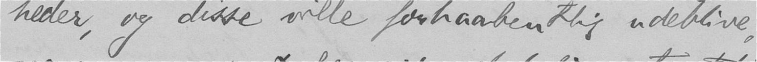heder, og disse ville forhaabentlig udeblive,
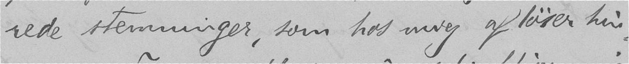rede stemninger, som hos mig afløser hin-
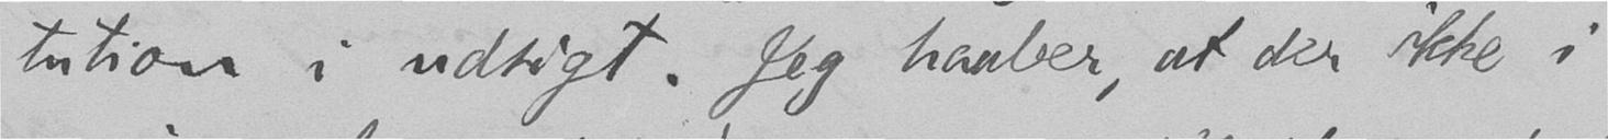tution i udsigt. Jeg haaber, at der ikke i
